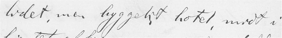lidet, men hyggeligt hotel, midt i
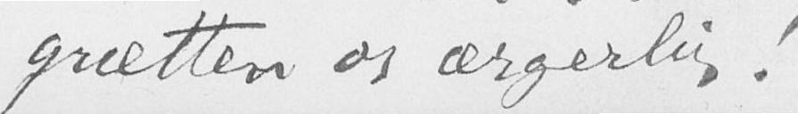grætten og ærgerlig!
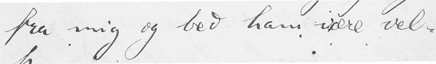fra mig og bed ham være vel-
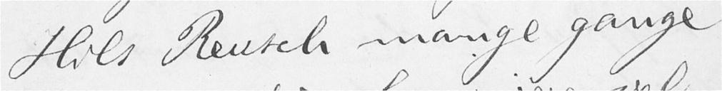Hils Reusch mange gange
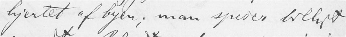hjertet af byen; man spiser billigt
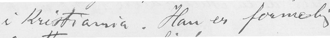i Kristiania. Han er formelig
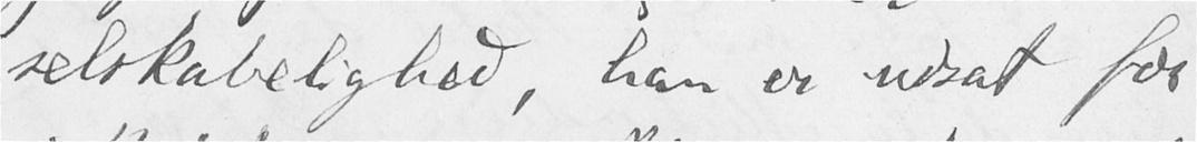selskabelighed, han er udsat for
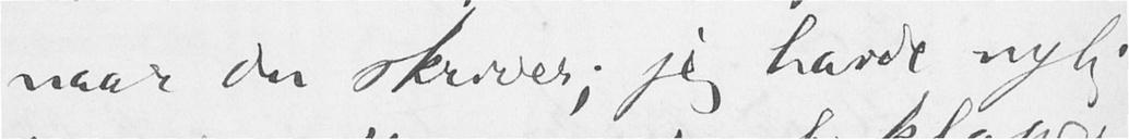naar du skriver; jeg havde nylig
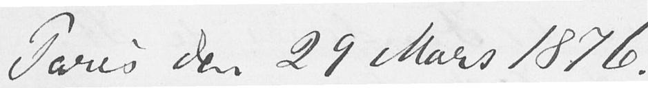Paris den 29 Mars 1876.
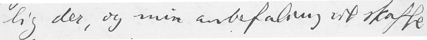lig der, og min anbefaling vil skaffe
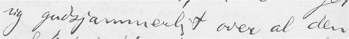sig gudsjammerligt over al den
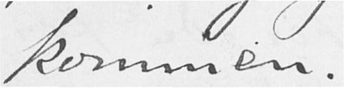kommen.
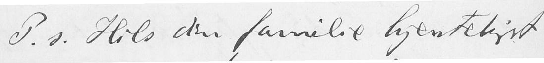P.s. Hils din familie hjerteligst
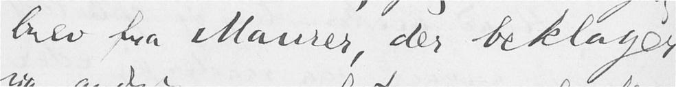brev fra Maurer, der beklager
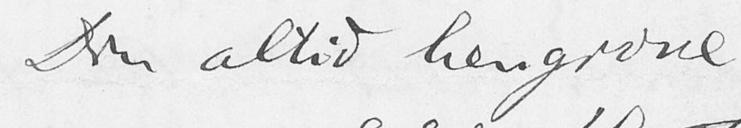Din altid hengivne
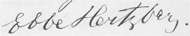Ebbe Hertzberg.
